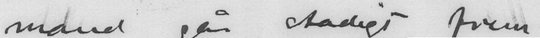mand går stadig frem
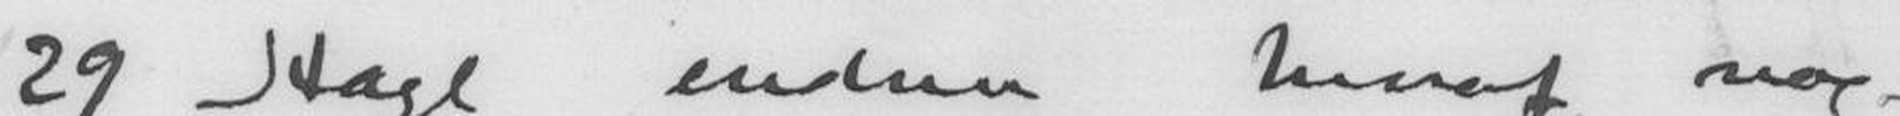29 Hagl endnu hvoraf nog
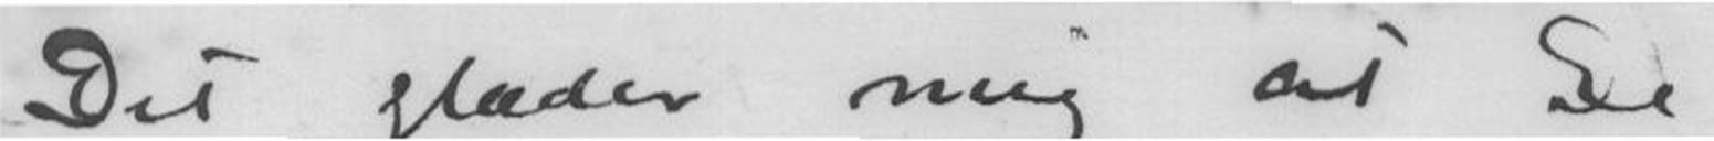Det glæder mig at De
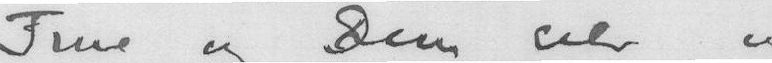Frue og Dem selv og
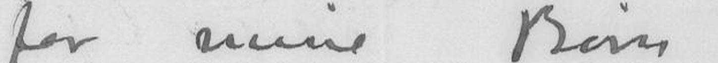for mine Børn
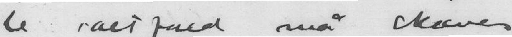le ialtfald må skæres
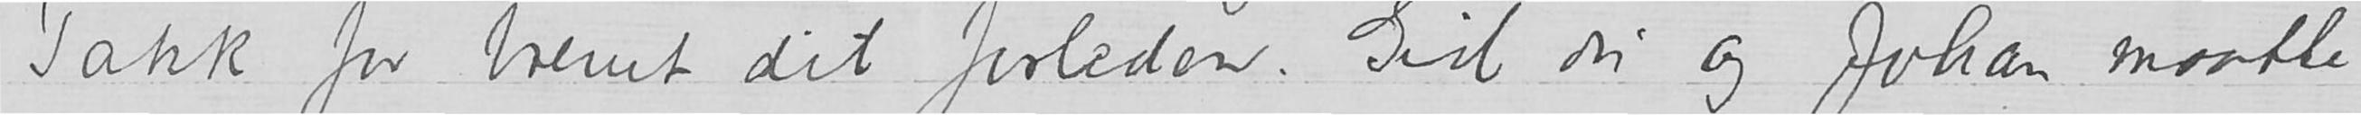Takk for brevet dit forleden.  Gid du og Johan maatte
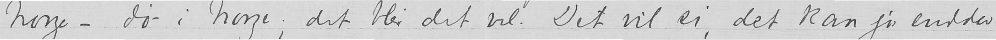Norge – dø i Norge, det blir det vel. Det vil si, det kan jo endda
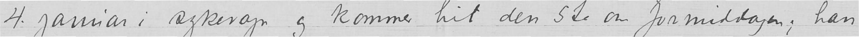4. januar i sykevogn og kommer hit  den 5te om formiddagen, han
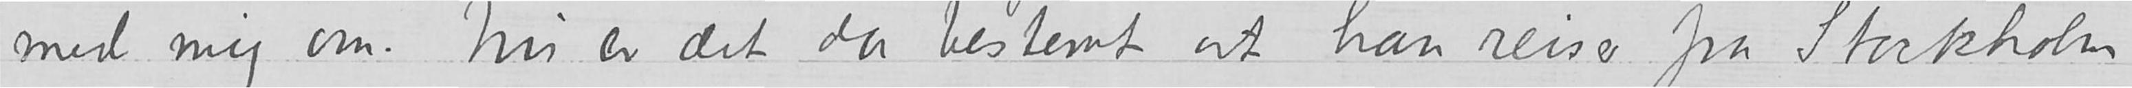med mig om.  Nu er det da bestemt at han reiser fra Stockholm
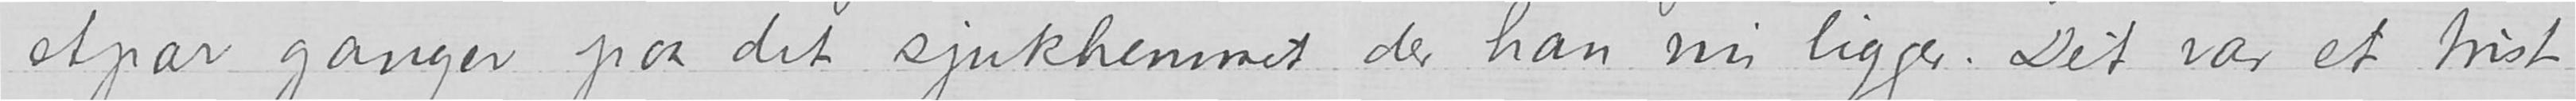et par ganger paa det sjukhemmet der han nu ligger. Det var et trist
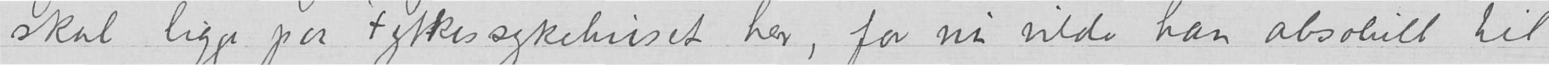skal ligge paa Fylkessykehuset her, for nå vilde han absolutt til
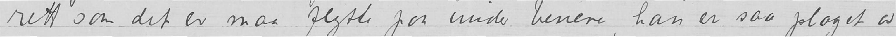rett som det er maa flytte <paa under benene>, han er saa plaget av
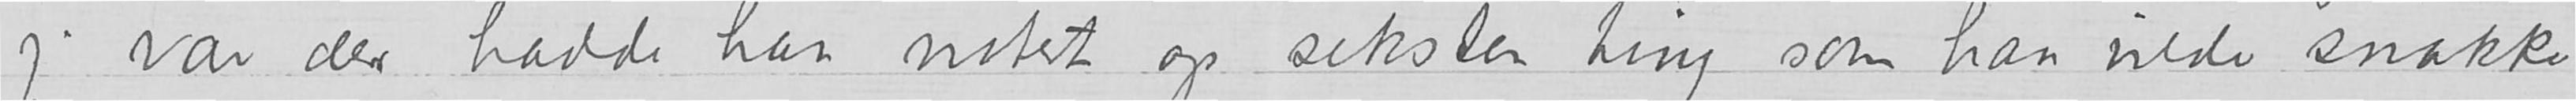jeg var der hadde han notert op seksten ting som han vilde snakke
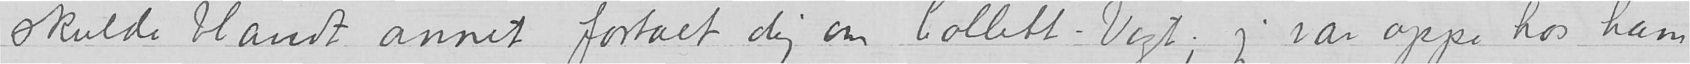skulde blandt annet fortalt dig om Collett-Vogt, jeg var oppe hos ham
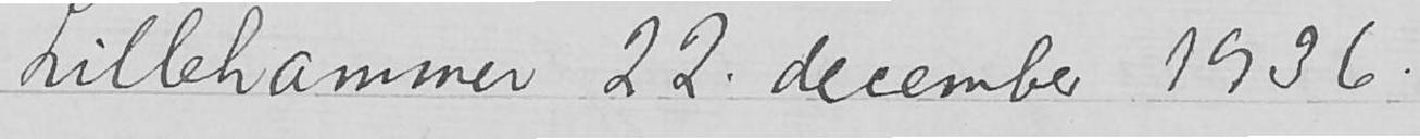Lillehammer 22. descember 1936
